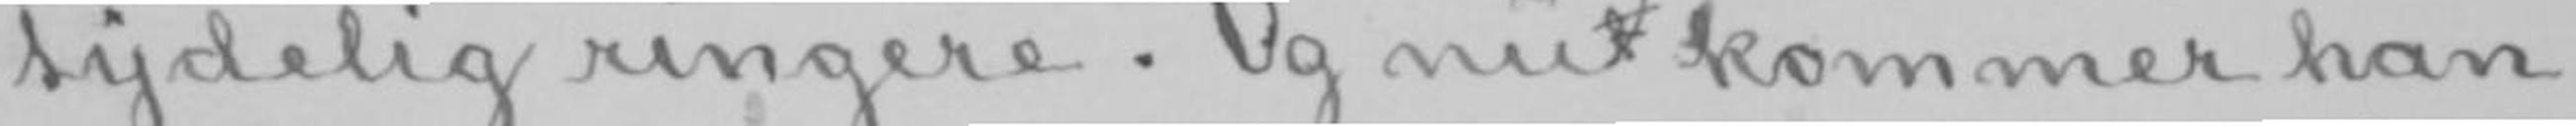tydelig ringere. Og nut kommer han
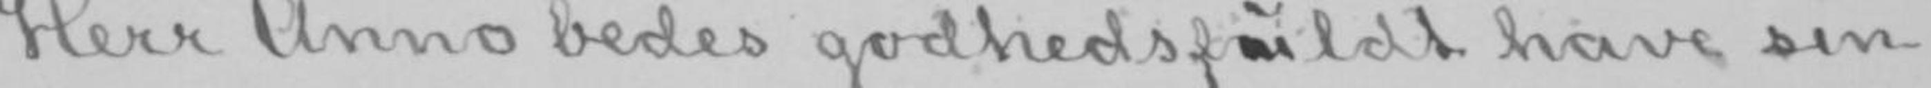Herr Anno bedes godhedsfuldt have sin
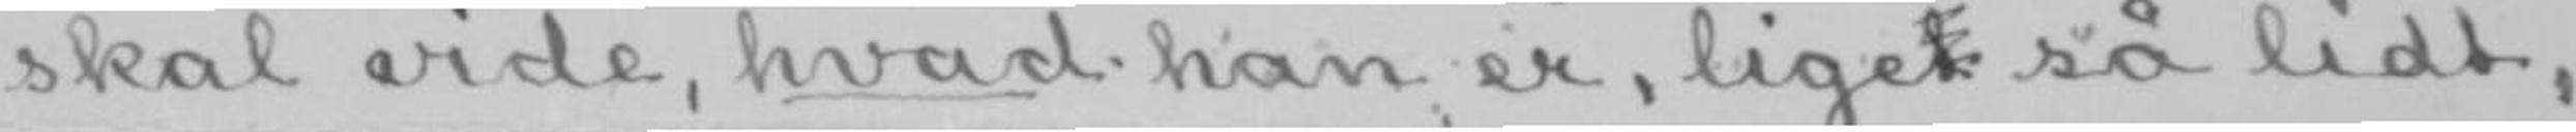skal vide, hvad han er, liget så lidt,
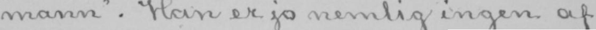mann». Han er jo nemlig ingen af
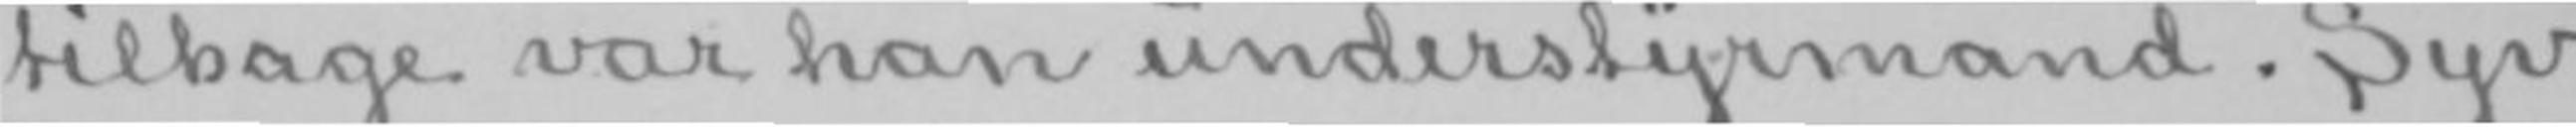tilbage var han understyrmand. Syv
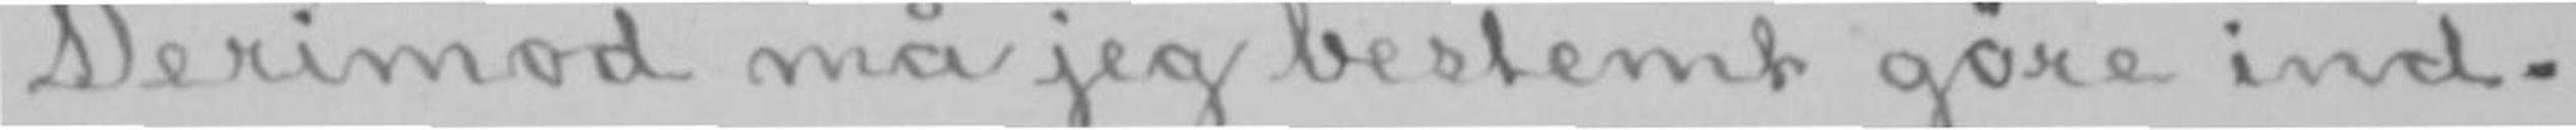Derimod må jeg bestemt gøre ind-
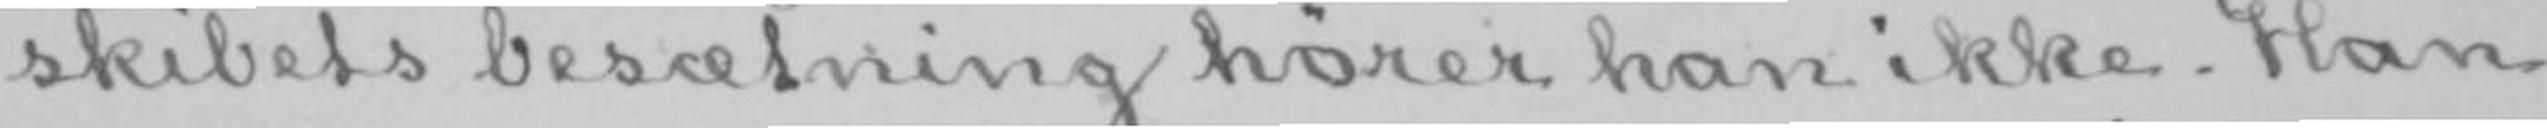skibets besætning hører han ikke. Han
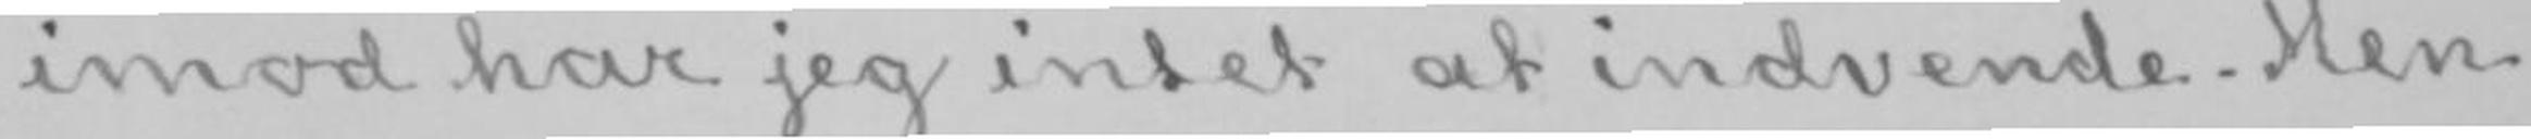imod har jeg intet at indvende. Men
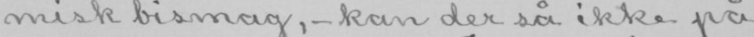misk bismag,- kan der så ikke på
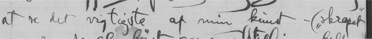at se det vigtigste af min kunst - ("skrapet"
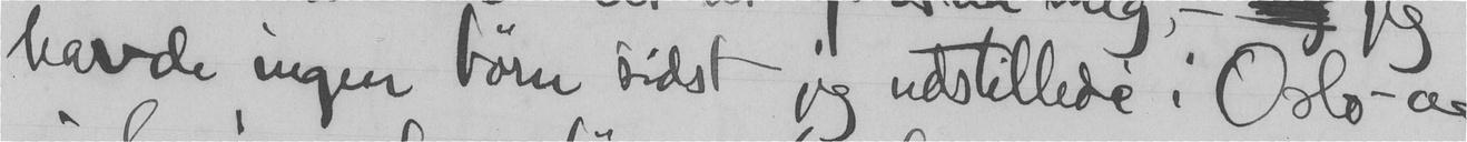havde ingen børn sidst jeg udstillede i Oslo - og
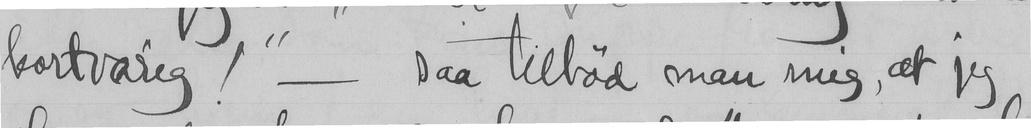kortvarig!" - saa tilbød man mig, at jeg
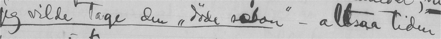jeg vilde tage den "døde sabon", - altsaa tiden
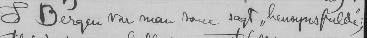I Bergen var man sone sagt "hensynsfulde";
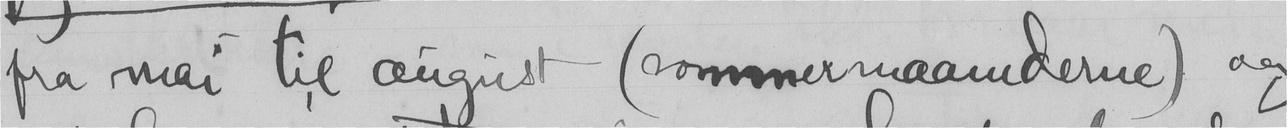fra mai til august (sommermaanderne) og
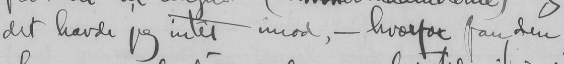det havde jeg intet imod, - hvorfor fan den
